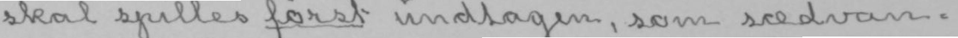skal spilles først undtagen, som sædvan-
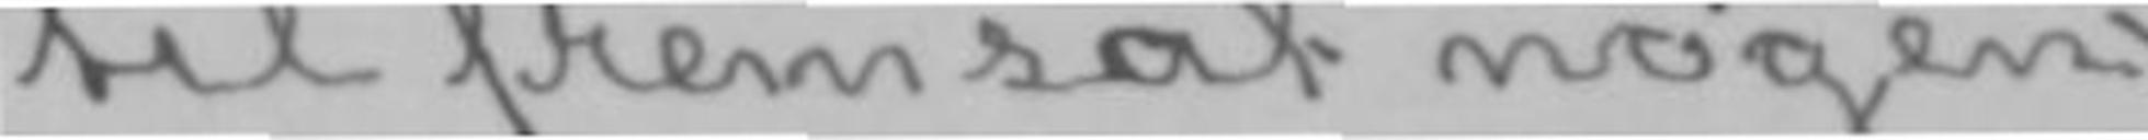til fremsat nogen
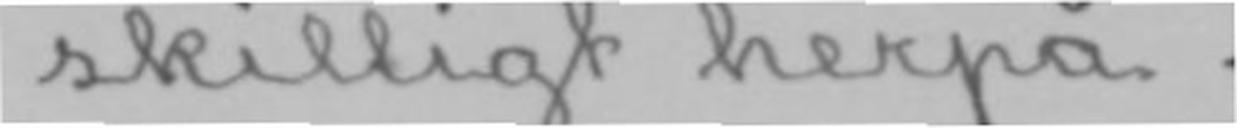skilligt herpå.
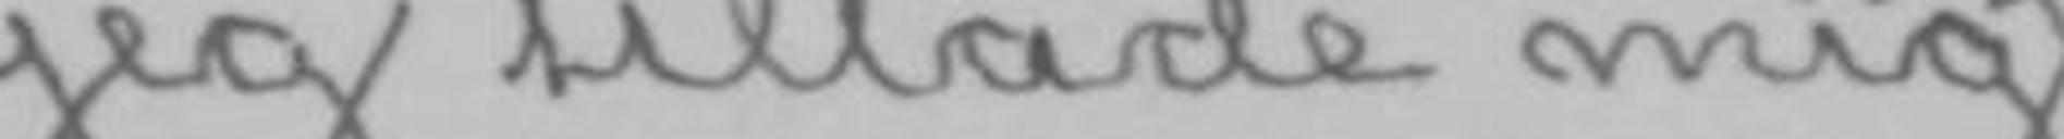jeg tillade mig
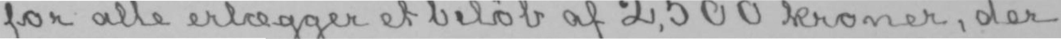for alle erlægger et beløb af 2,500 kroner, der
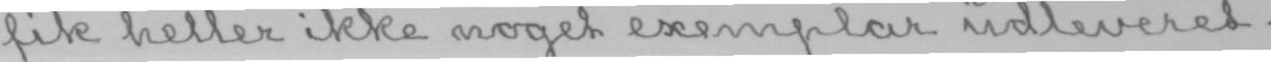fik heller ikke noget exemplar udleveret.
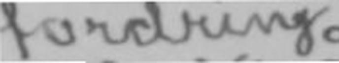fordring
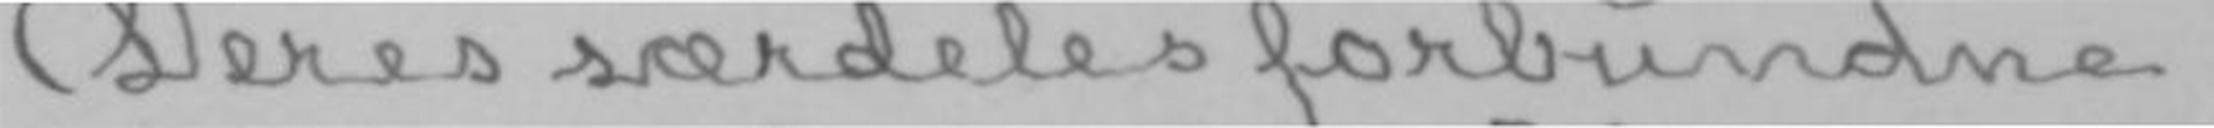Deres særdeles forbundne
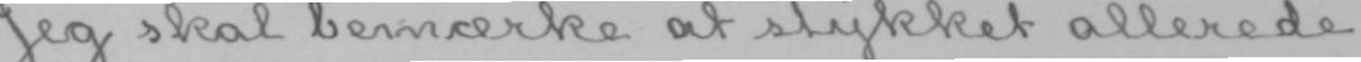Jeg skal bemærke at stykket allerede
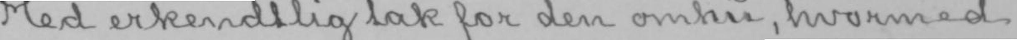Med erkendtlig tak for den omhu, hvormed
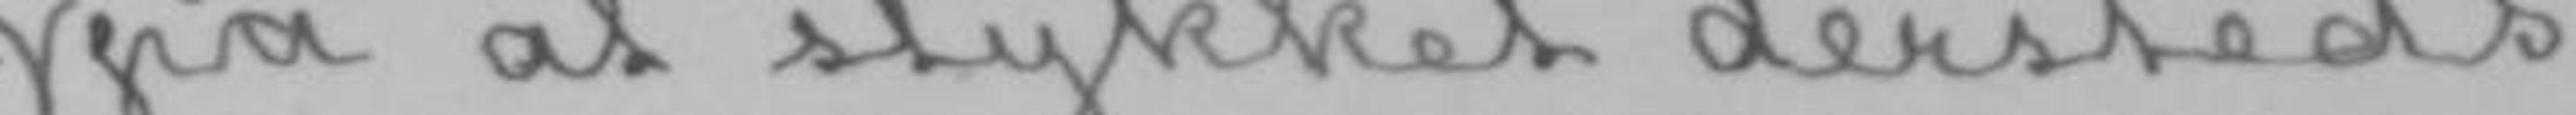på at stykket dersteds
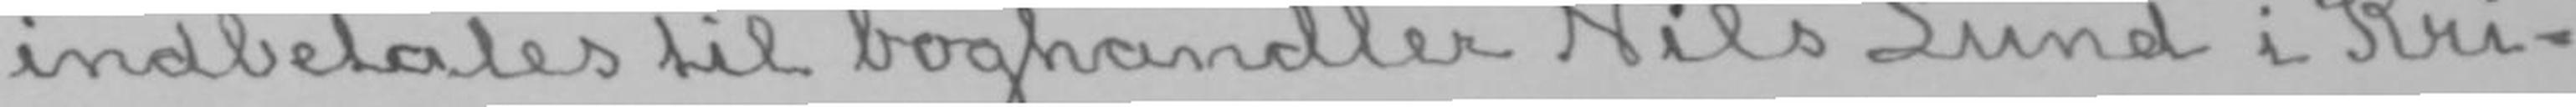indbetales til boghandler Nils Lund i Kri-
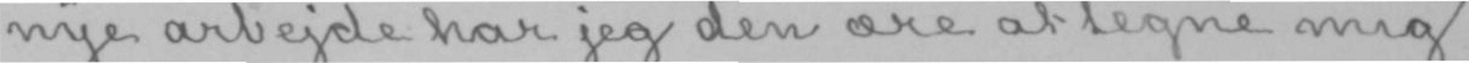nye arbejde har jeg den ære at tegne mig
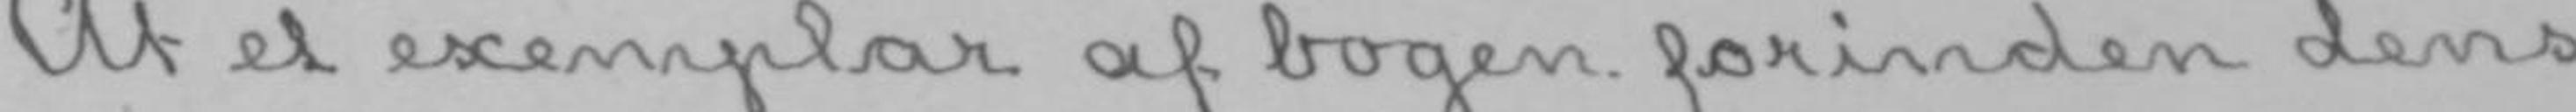At et exemplar af bogen forinden dens
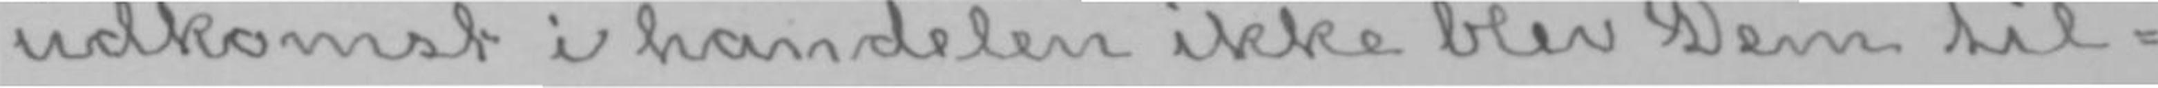udkomst i handelen ikke blev Dem til-
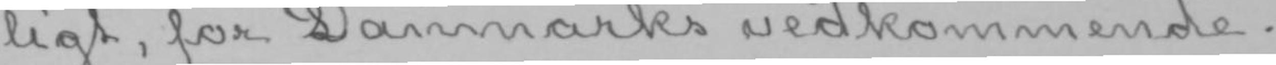ligt, for Danmarks vedkommende.
